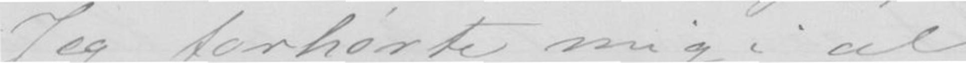Jeg forhørte mig i al
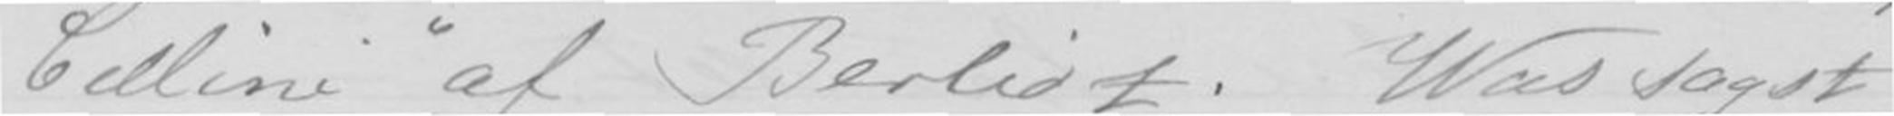Cellini af Berlioz. Was sagst
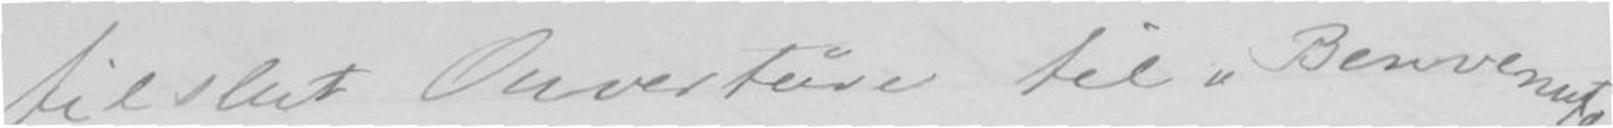tilslut Ouvertüre til Benvenuto
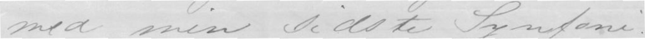med min sidste Symfoni.
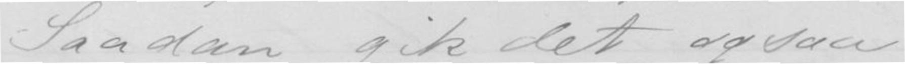Saadan gik det ogsaa
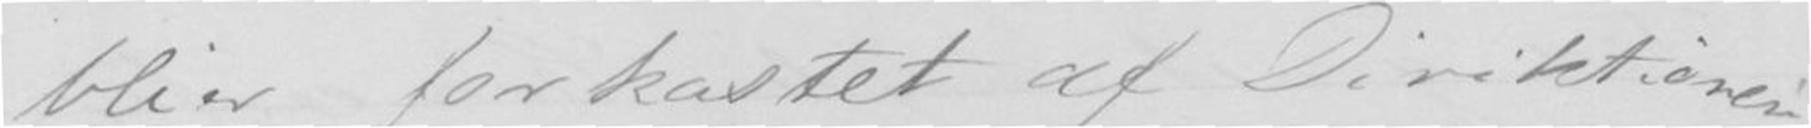blier forkastet af Diriktionen.
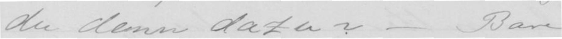du denn dazu? - Bare
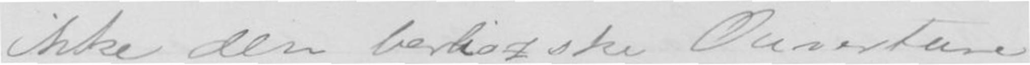ikke den berliozske Ouverture
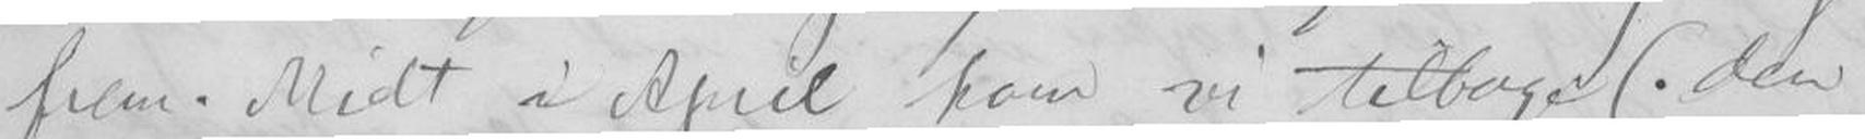frem. Midt i April kom vi tilbage (den
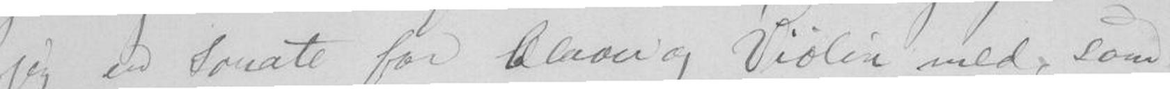jeg en Sonate for Claver og Violin med, som
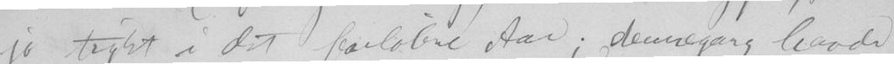jo trykt i det paaløbne Aar; dengang havde
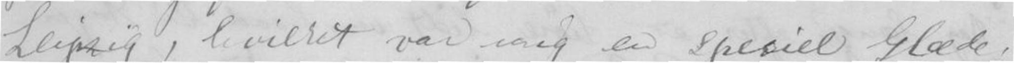Leipzig, hvilket var mig en spesiel Glæde;
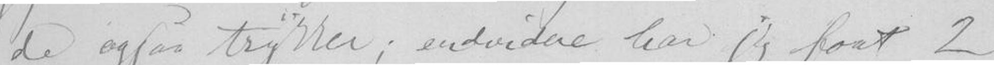de ogsaa trykker; endvidere har jeg faat 2
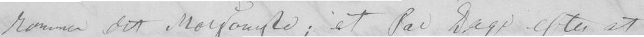kommer det Morsomste; et Par Dage efter at
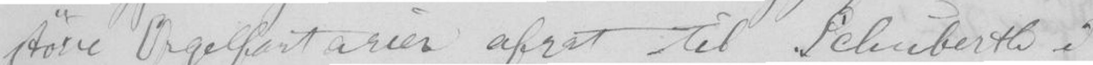større Orgelfantasier afsat til Schubert i
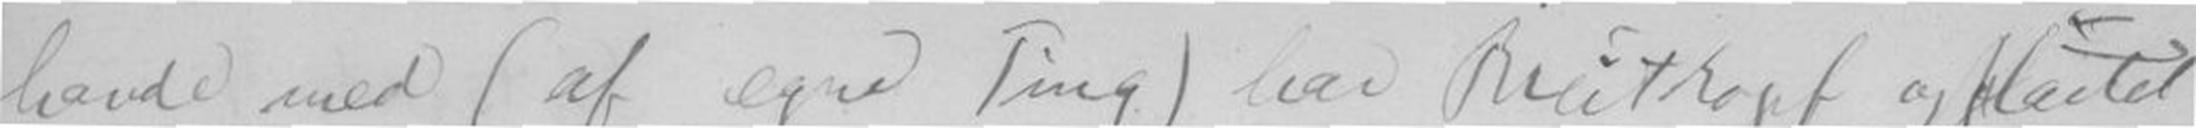havde med (af egne Ting) har Breitkopf og Härtel
